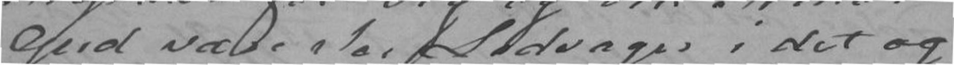Gud være Jer Ledsager i det og
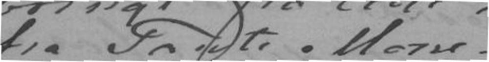fra Tante Mone.
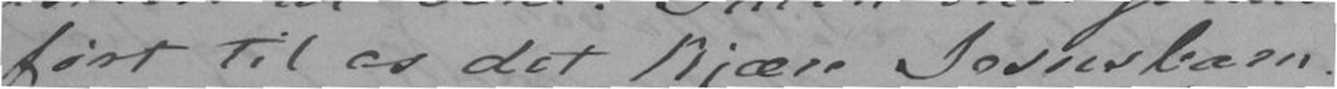ført til os det kjære Jesusbarn.
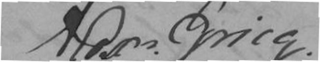Alexr Grieg.
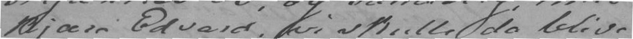kjære Edvard, vi skulle da blive
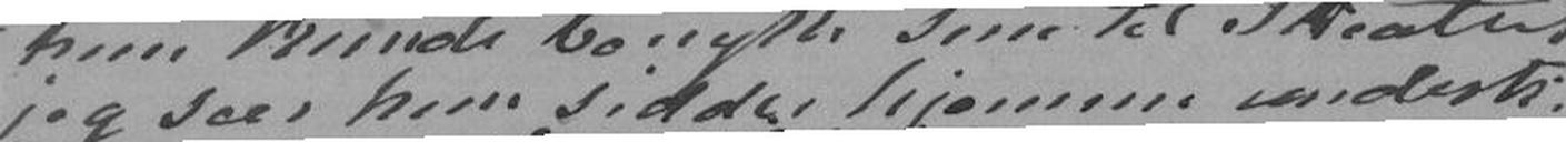jeg seer hun sidder hjemme underti-
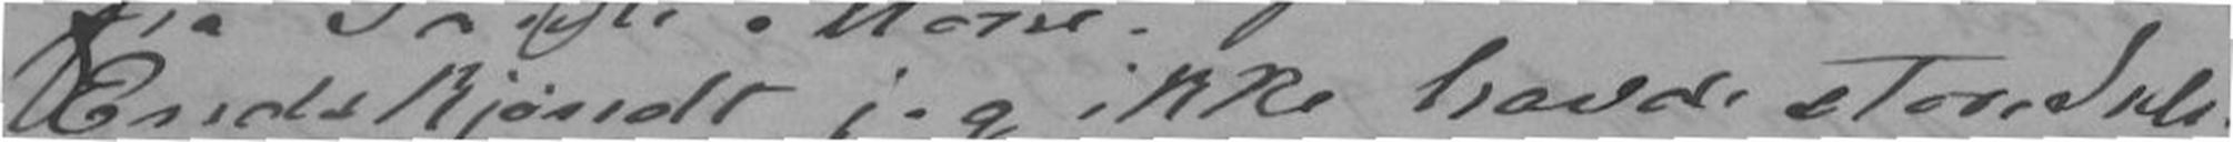Endskjøndt jeg ikke havde store Jule-
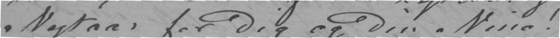Nytaar for Dig og Din Nina!
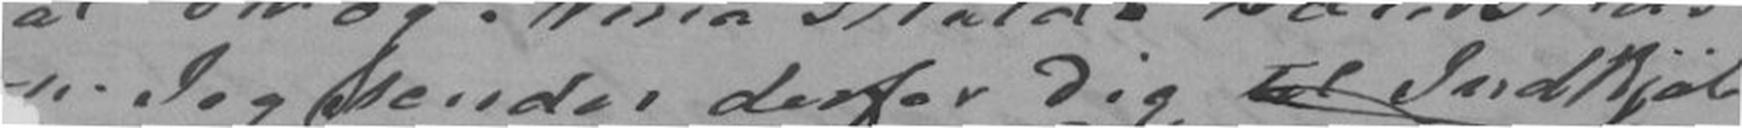Jeg sender derfor Dig til Indkjøb
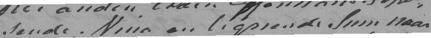sende Nina en lignende Sum naar
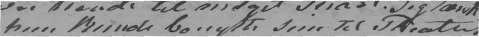hun kunde benytte sine til Theater.
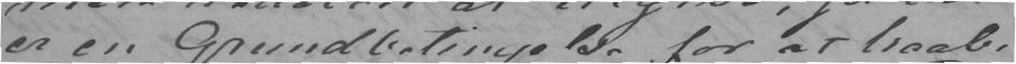er en Grundbetingelse for at haabe
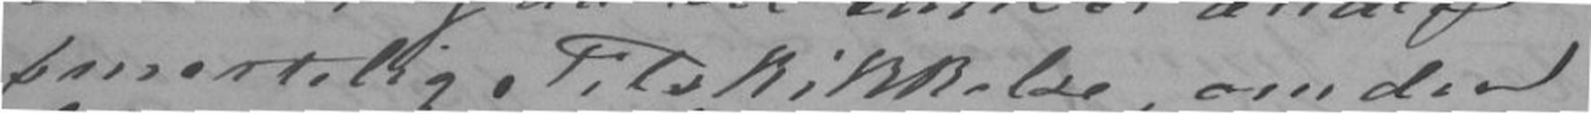smertelig Tilskikkelse, om den
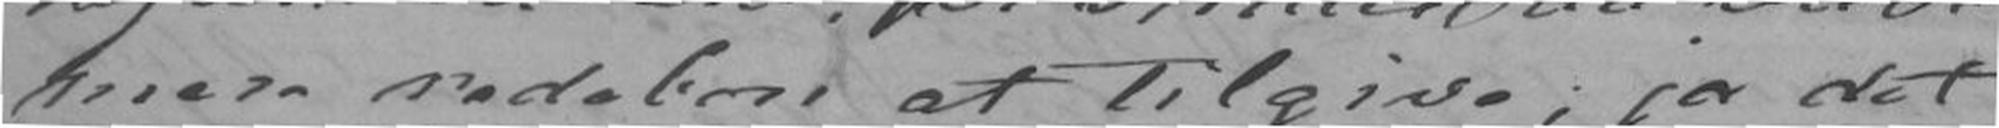mere redebon at tilgive; ja det
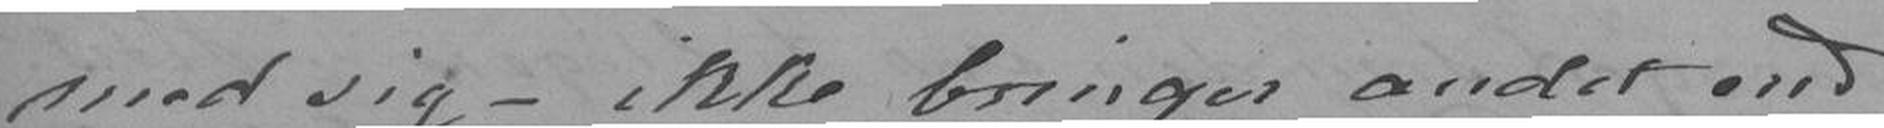med sig - ikke bringer andet end
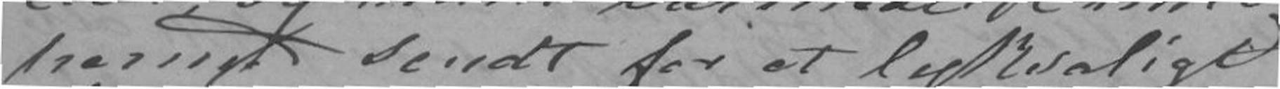hermed sendt for et lyksaligt
